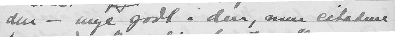den - mye godt i den, men citatene -
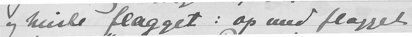og heiste flagget; op med flagget
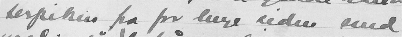terpiken fra for lenge siden med
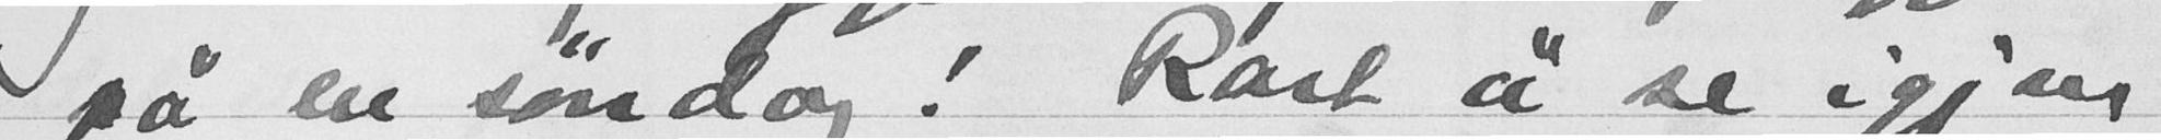på en søndag! Rart å se igjen
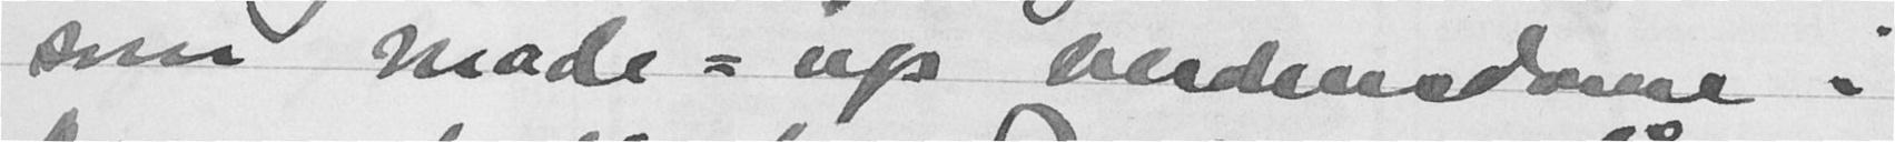som made-up berdensdame i
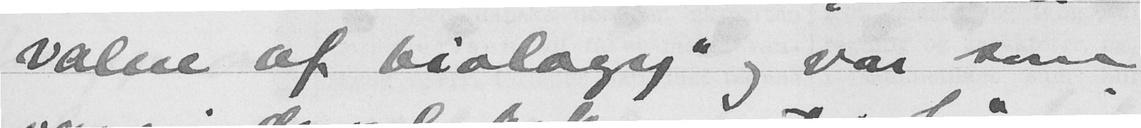Value of biology" og var som
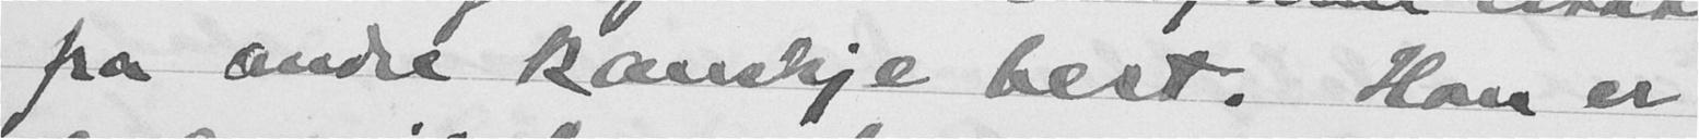fra andre kanskje best. Han er
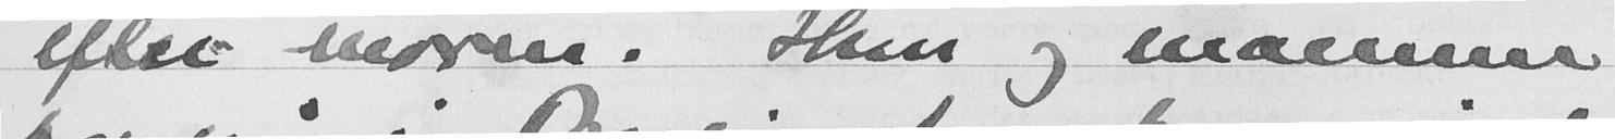efter moren. Hun og mannen
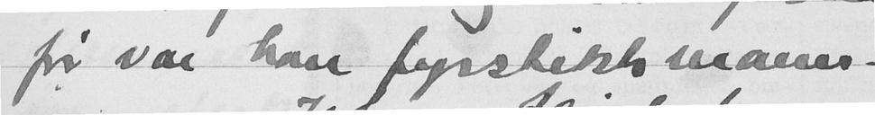før var han fyrstikkmann.
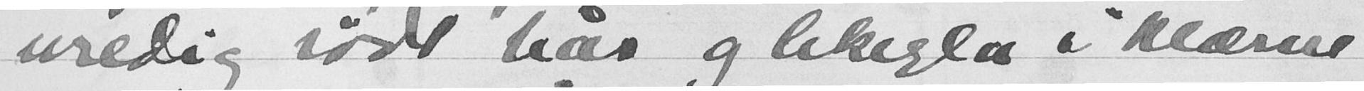uredig rødt hår og likegla i klærne
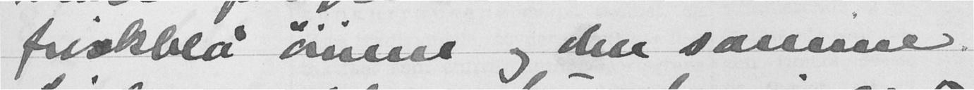friskblå øinene og den samme
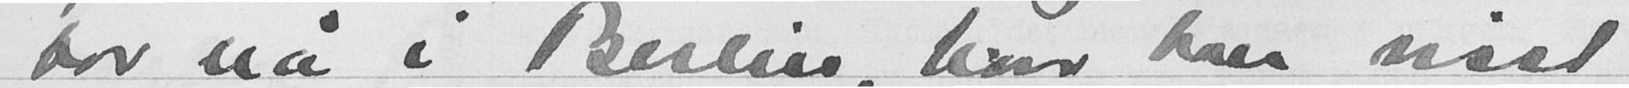bor nå i Berlin, hvor han visst
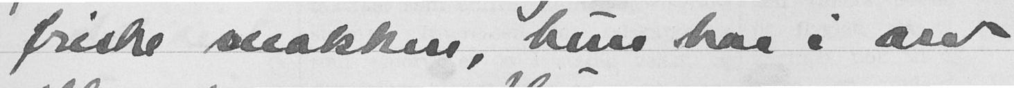friske snakken, hun har i arv
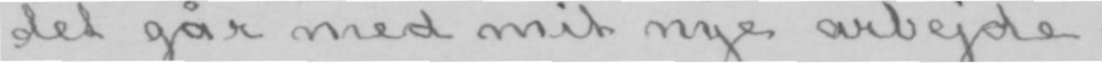det går med mit nye arbejde.
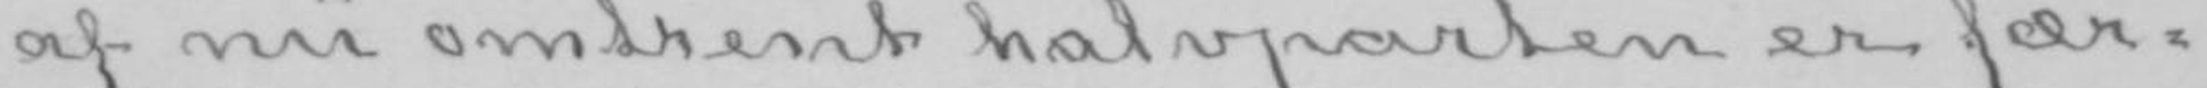af nu omtrent halvparten er fær-
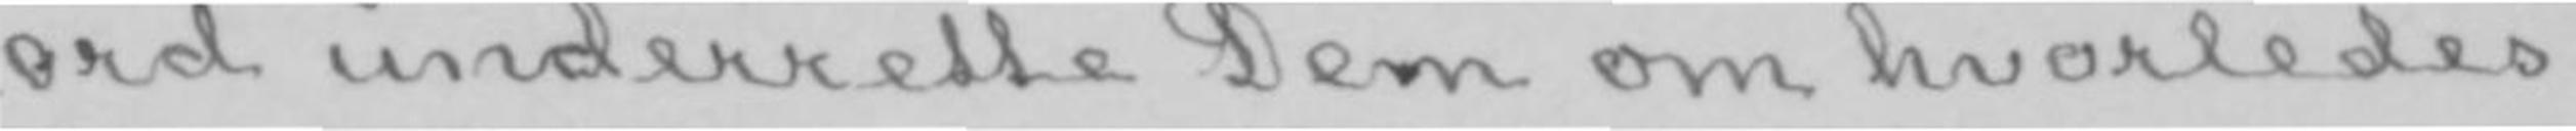ord underrette Dem om hvorledes
